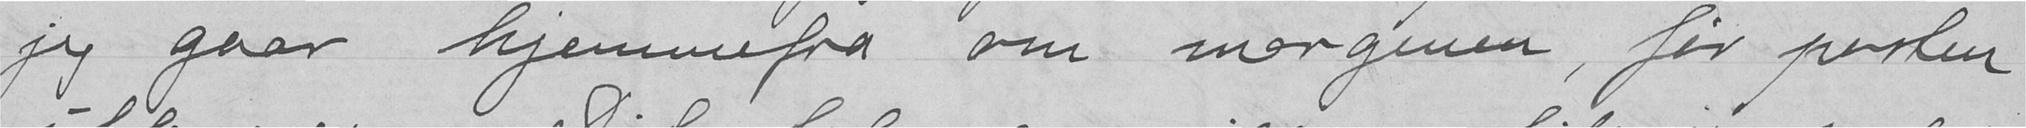jeg gaar hjemmefra om morgenen, før posten
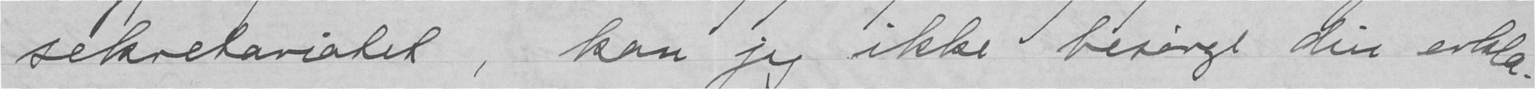sekretariatet, kan jeg ikke besørge din erklæ-
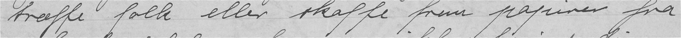træffe folk eller skaffe frem papirer fra
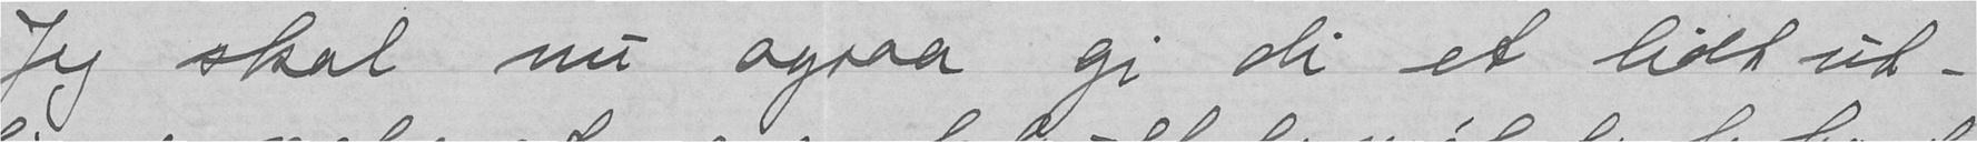Jeg skal nu ogsaa gi di et lidt ut-
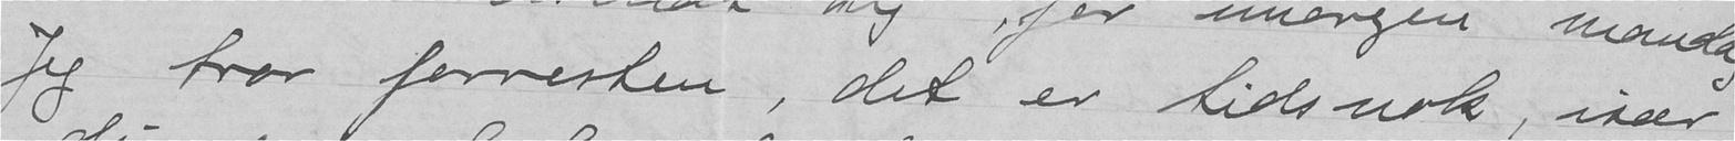Jeg tror forresten, det er tidsnok, især
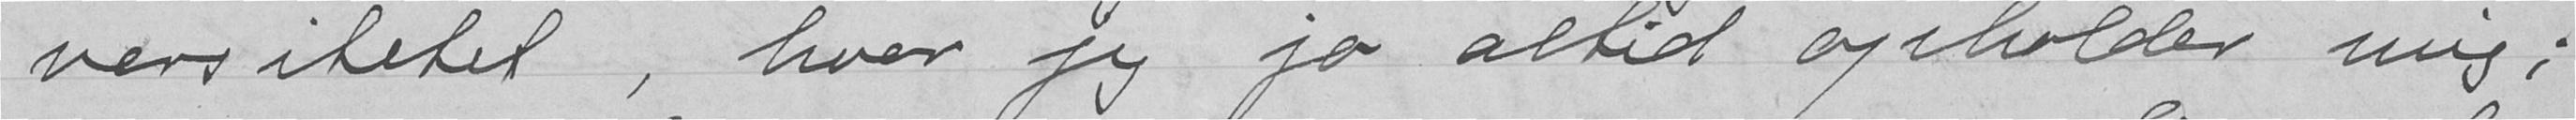versitetet, hver jeg jo altid opholder mig;
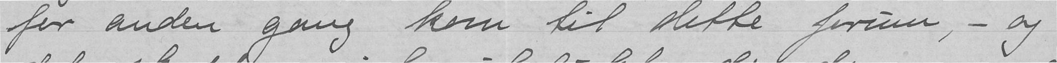for anden gang kom til dette forum, - og
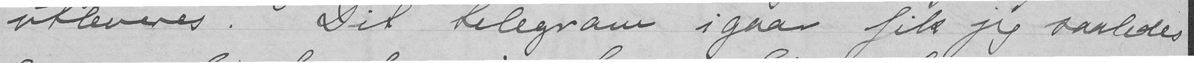utleveres. Dit telegram igaar fik jeg saaledes
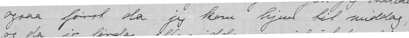ogsaa først da og kom hjem til middag,
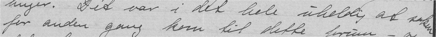linger. Det var i det hele uheldig at saken
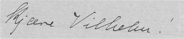Kjære Vilhelm!
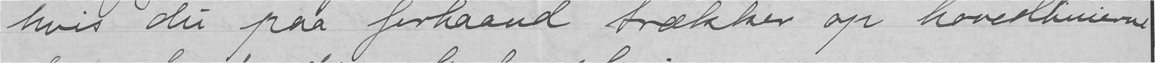hvis der paa forhaand trækker op hovedlinierne
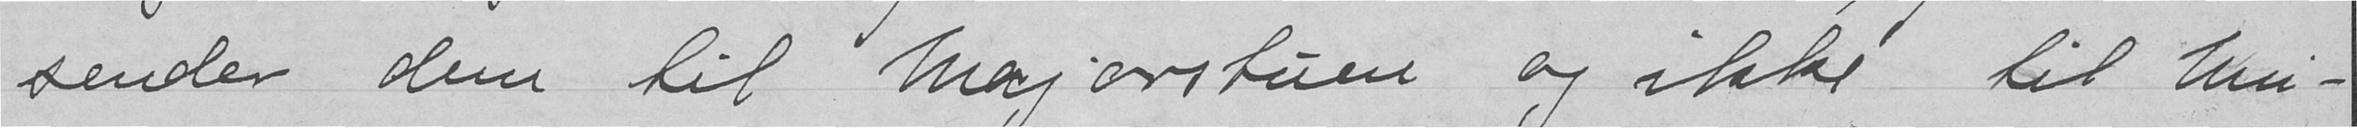sender dem til Majorstuen og ikke til Uni-
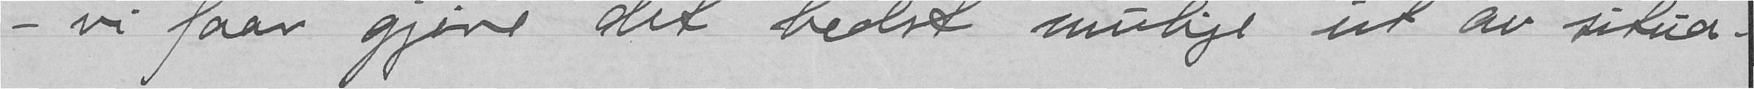- vi faar gjøre det bedst mulige ut av situa-
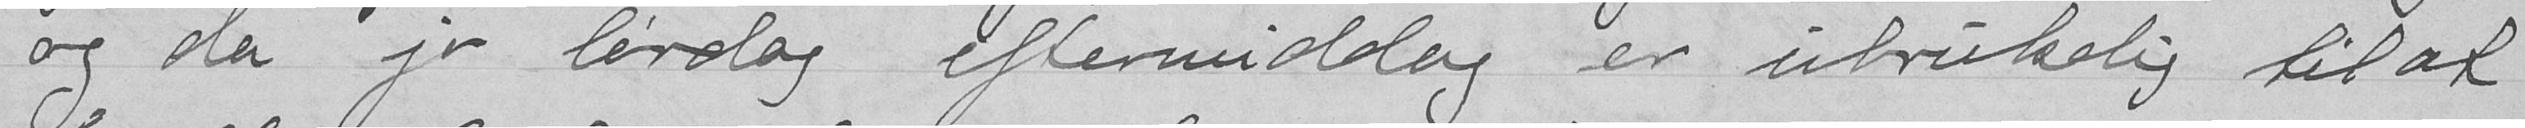og da jo lørdag eftermiddag er ubrukelig til at
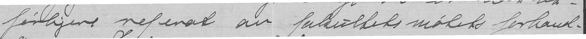førligere referat av fakultetsmøtets forhand-
